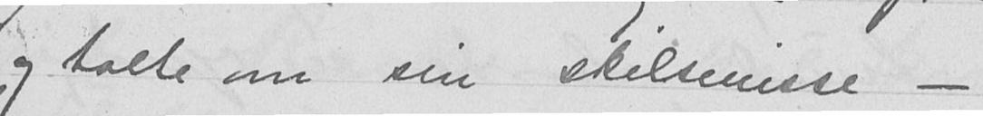og talte om sin skilsmisse -
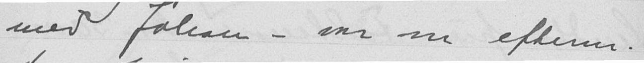med Johan - var om efterm.
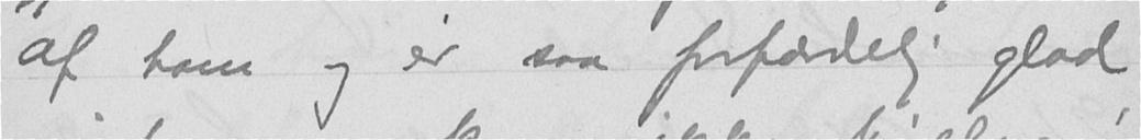af ham og er saa forfædelig glad
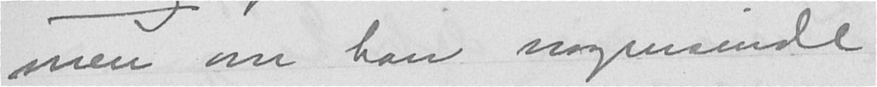men om han nogensinde
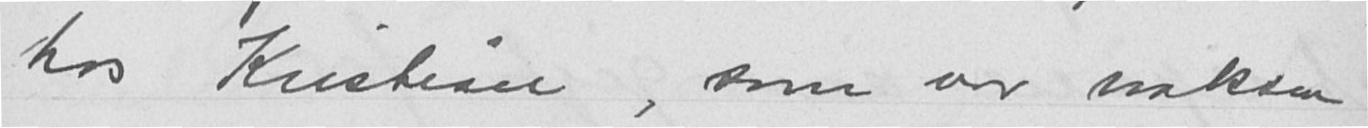hos Kristian, som var noksaa
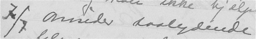7/7 Omsider saalydende
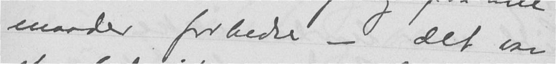maader forbedre - det var
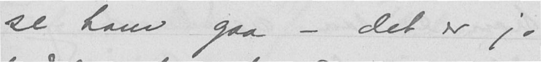se ham gaa - det er jo
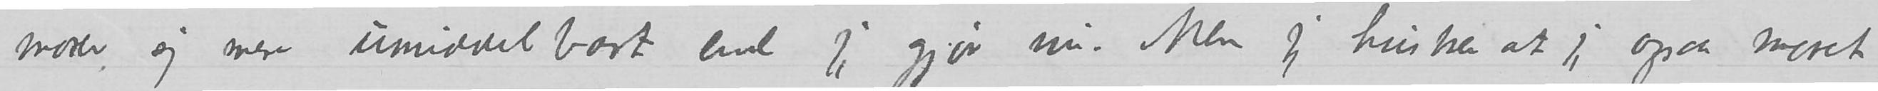morer seg mere umiddelbart end jeg gjør nu. Men jeg husker at jeg ogsaa moret
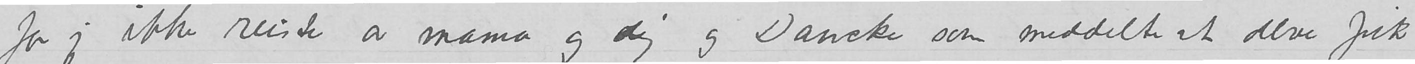for jeg ikke reiste av mama og dig og Daucke som meddelte at dere fik
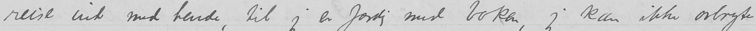reise ind med hende, til jeg er færdig med boken, jeg kan ikke avbryte
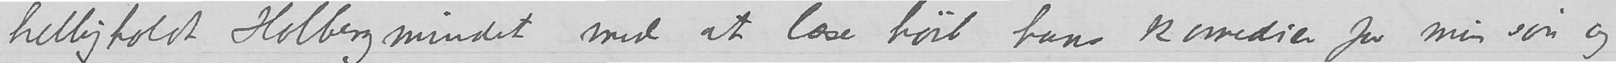helligholdt Holbergmindet med at læse høit hans komedier for min søn og
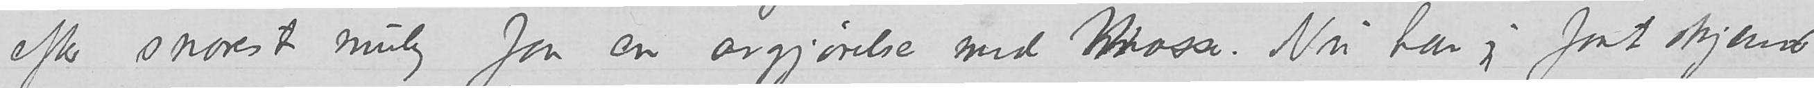efter snarest mulig faa en avgjørelse med Mosse. Nu har jeg faat skjænd
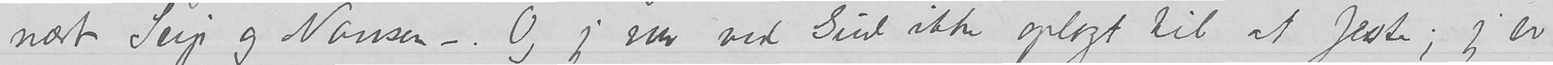nært Seip og Nansen -. Og jeg var ved Gud ikke oplagt til at feste; jeg er
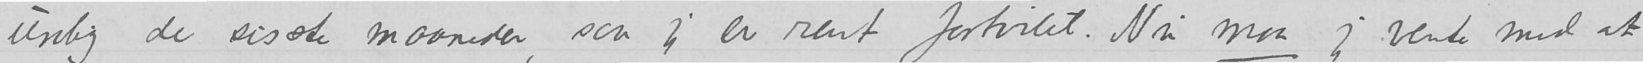urolig de sisste maaneder, saa jeg er rent fortvilet. Nu maa jeg vente med at
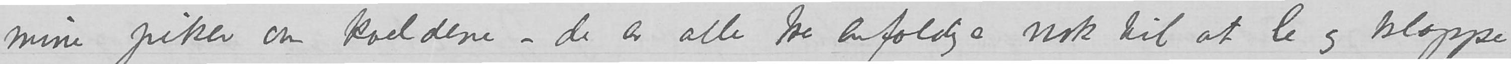mine piker om kveldene - de er alle tre enfoldige nok til at le og klappe
